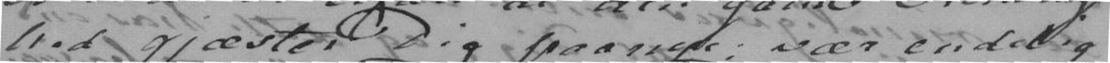hed gjæster dig panye; vær endelig
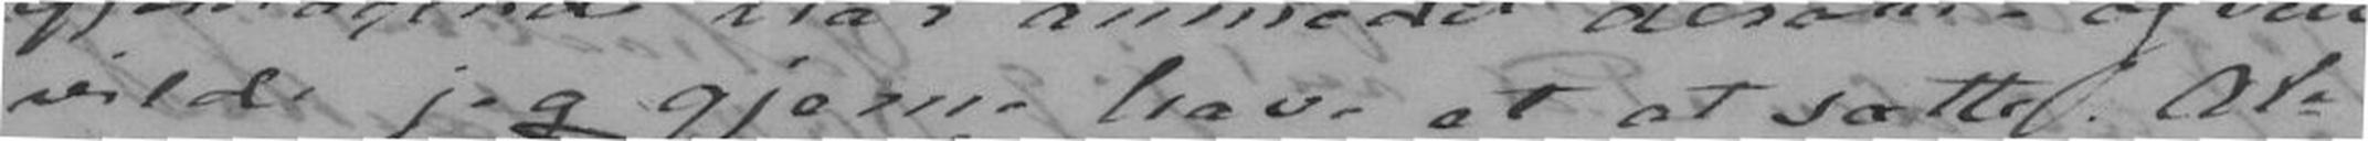vilde jeg gjerne have et at sætte i Al-
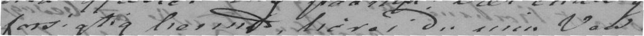forsigtig hermed hører Du min Ven?
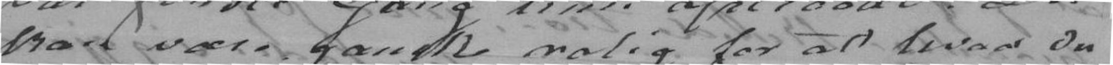kan være ganske rolig for at hvad Du
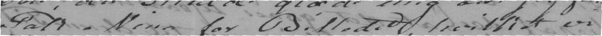Tak Nina for Billede, hvilket vi
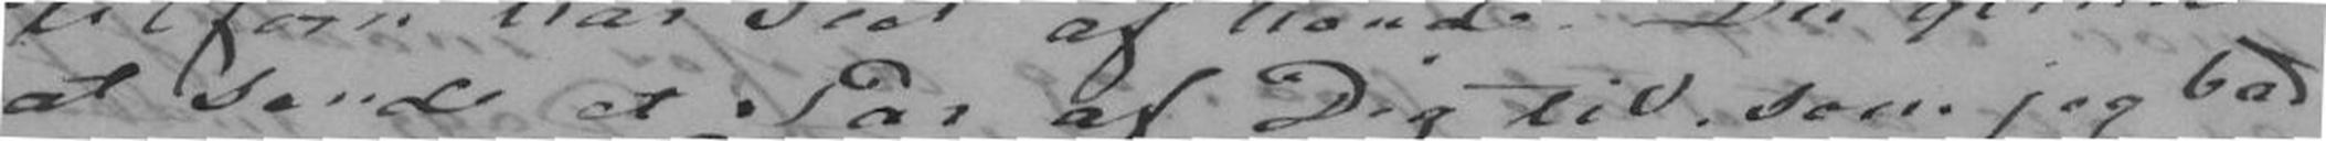at sende et Par af Dig til som jeg bad
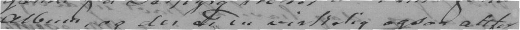Album, og der er Du virkelig ogsaa altfor
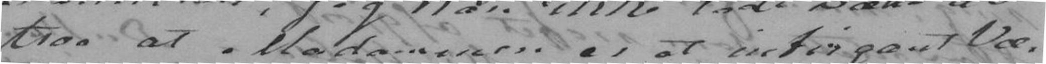troe at Madammen er et intrigant Væ-
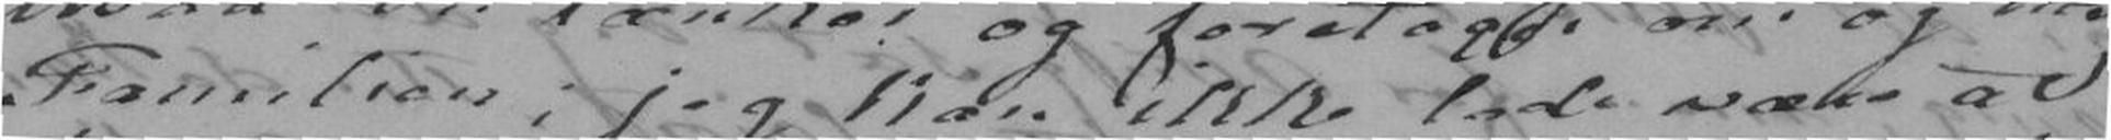Familien; jeg kan ikke lade være at
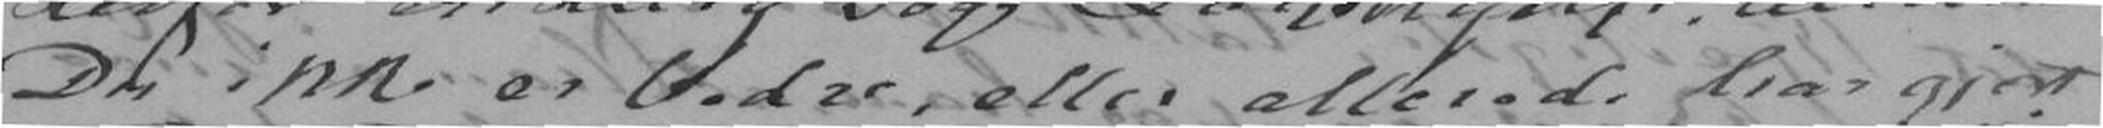Du ikke er bedre, eller allerede har gjort
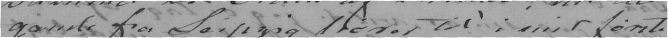gamle fra Leipzig hører til i mit første
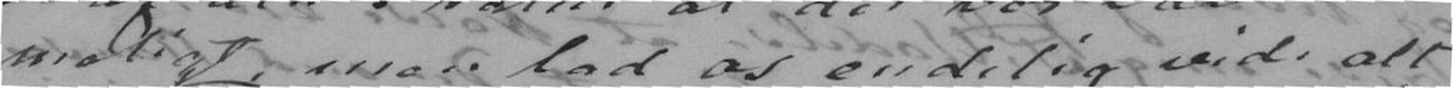meligt, men lad os endelig vide alt
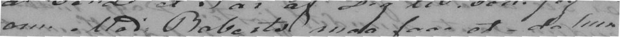om. Mad. Roberts maa faae et da hun
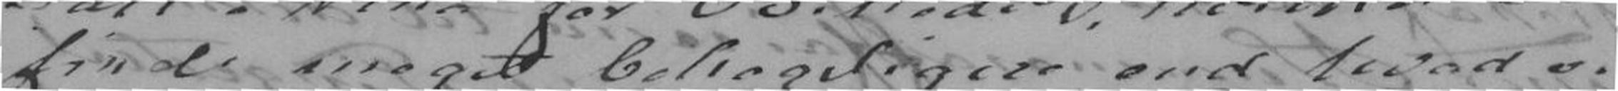finde meget behageligere end hvad vi
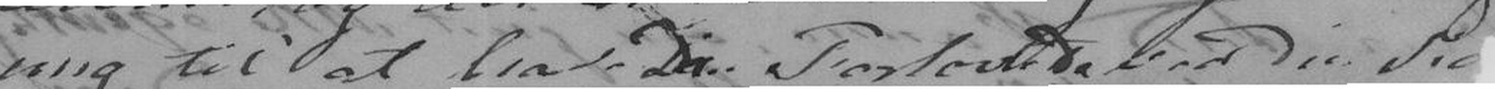ung til at have Din Forlovede ved Din Si
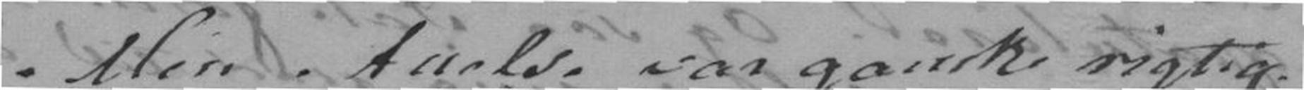Min Anelse var ganske rigtig
